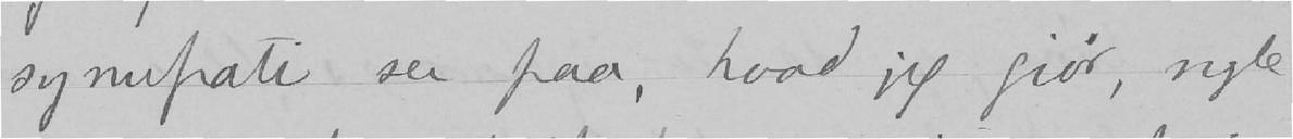sympati ser paa, hvad jeg giør, nogle
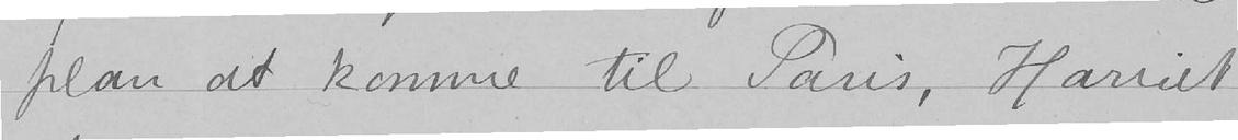plan at komme til Paris, Harriet
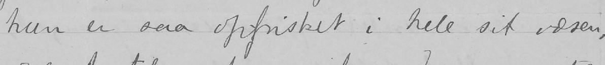hun er saa opfrisket i hele sit væsen,
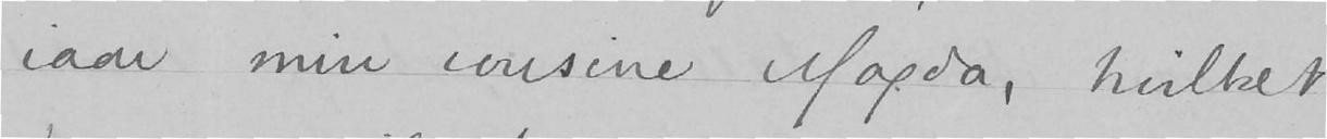iaar mine cousine Magda, hvilket
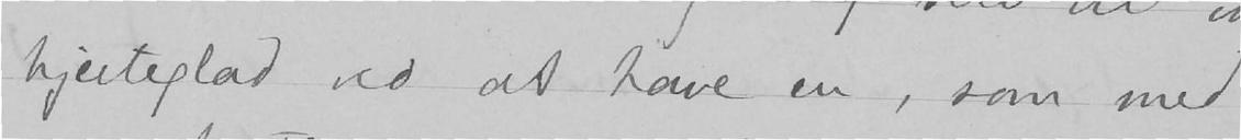hjerteglad ved at have en, som med
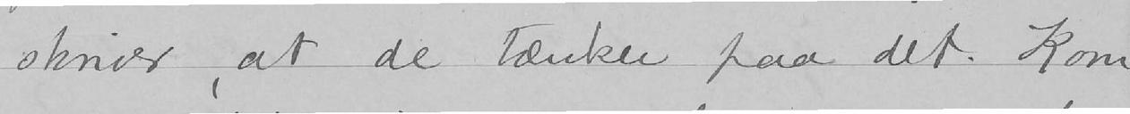skriver, at de tænker paa det. Kom
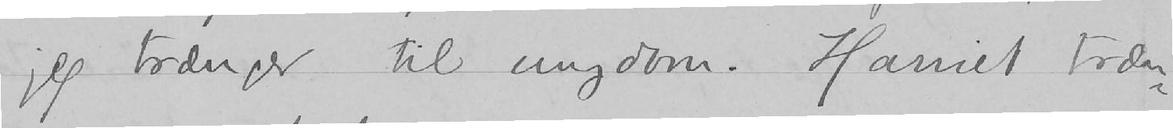jeg trænger til ungdom. Harriet træn
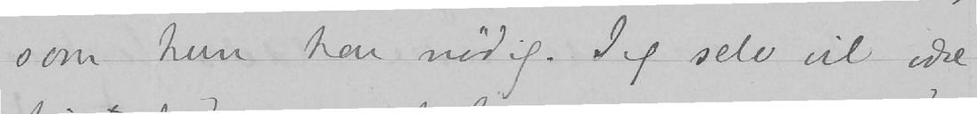som hun har nødig. Jeg selv vil være
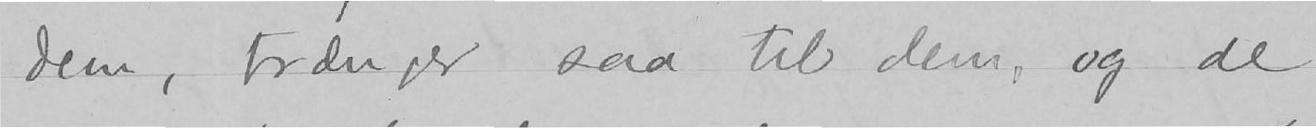dem, trænger saa til dem, og de
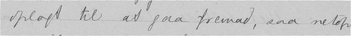oplagt til at gaa fremad, saa netop
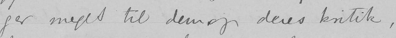ger meget til dem og deres kritik,
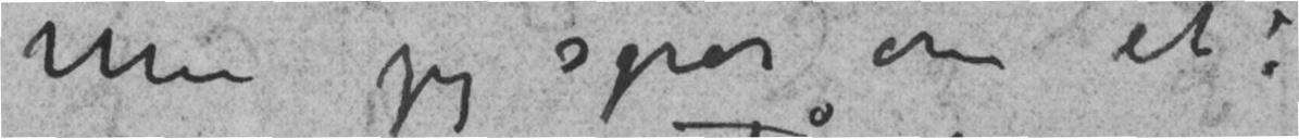Men jeg spør om et:
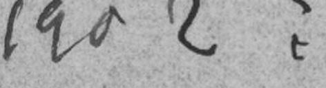1902?
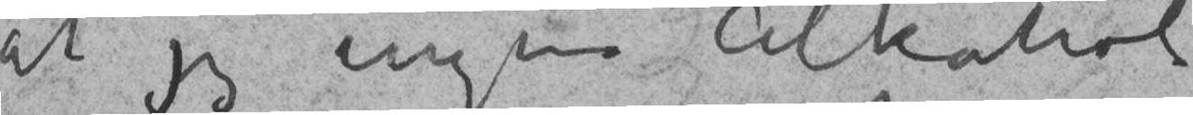at jeg ingen Alkohol
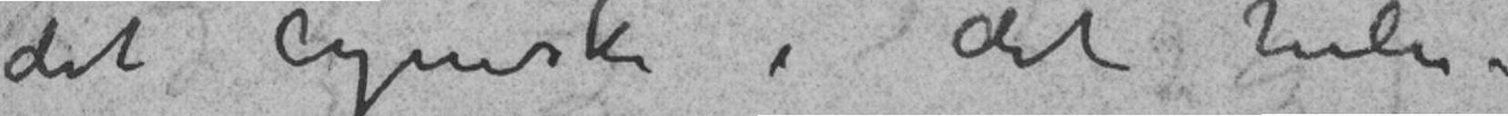det cyniske i det hele –
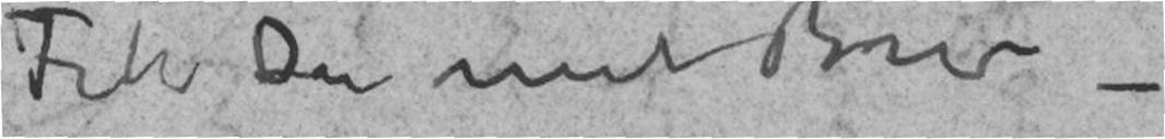Fik Du mit Brev –
